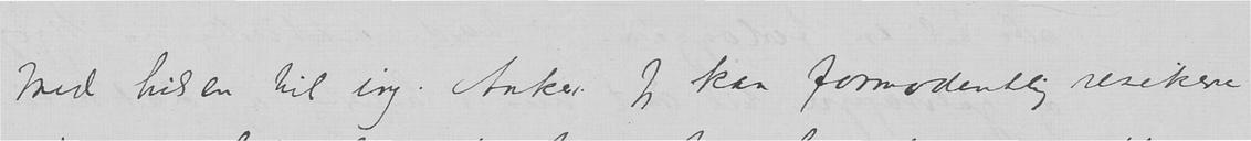Med hilsen til ing. Anker. Jeg kan formodentlig <risikere>
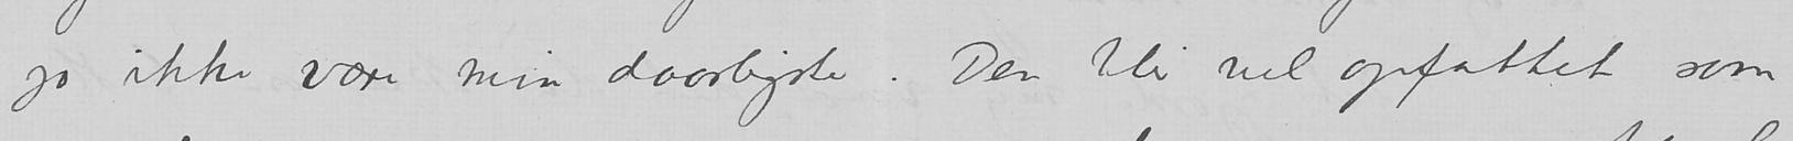jo ikke være min daarligste. Den blir vel opfattet som
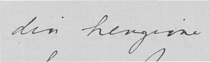din hengivne
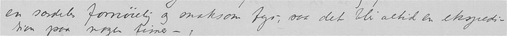er en særdeles fornøielig og snaksom fyr, saa det blir altid en ekspedi-
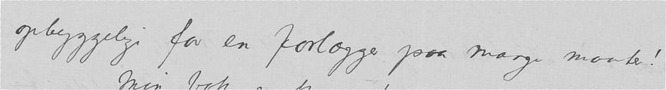opbyggelig for en forlægger paa mange maater!
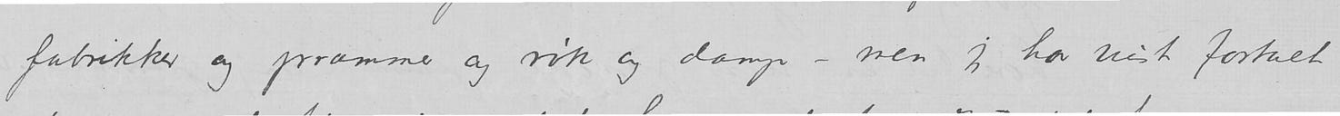fabrikker og prammer og røk og damp – men jeg har vist fortalt
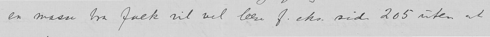en masse bra folk vil vel læse f.eks. side 205 uten at
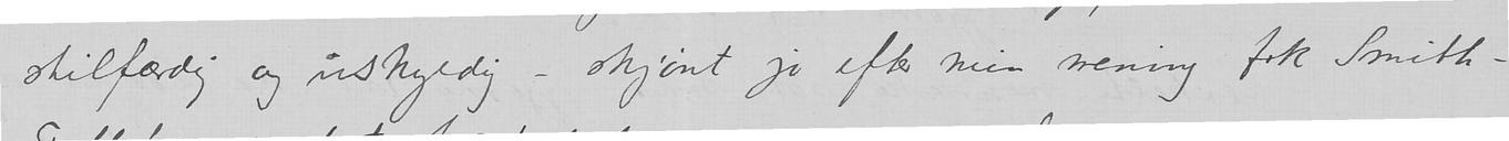stilfærdig og uskyldig – skjønt jo efter min mening frk Smith-
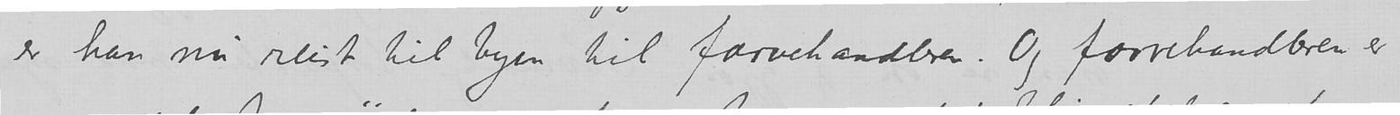er han nu reist til byen til farvehandleren. Og farvehandleren
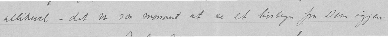allikevel – det var saa morsomt at se et livstegn fra Dem igjen.
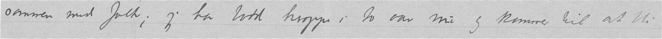sammen med folk; jeg har bodd heroppe i to aar nu og kommer til at bli
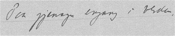Paa gjensyn engang i verden,
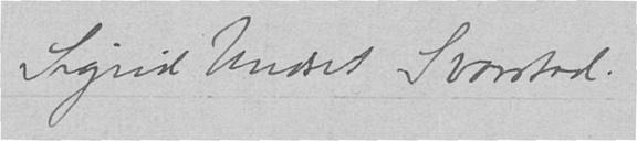Sigrid Undset Svarstad.
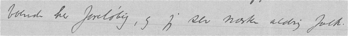boende her foreløbig, og jeg ser næsten aldrig folk.
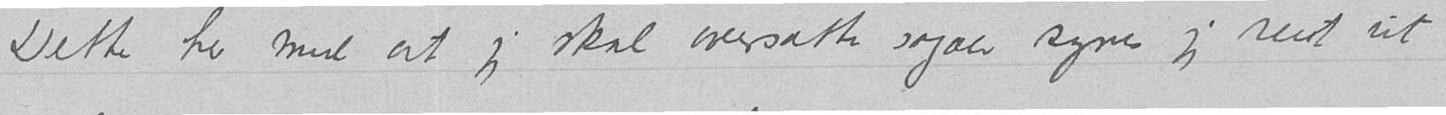Dette her med at jeg skal oversatte sagaer synes jeg rent ut
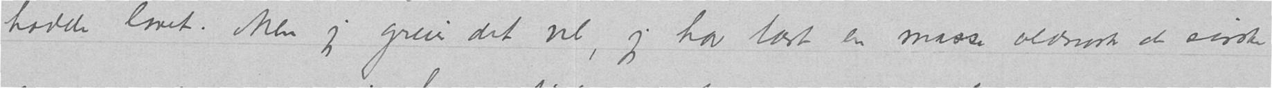hadde lovet. Men jeg greier det vel, jeg har læst en masse oldnorsk de siste
